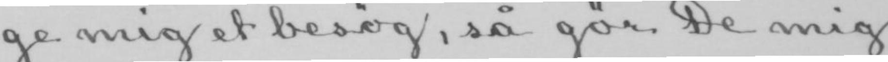ge mig et besøg, så gør De mig
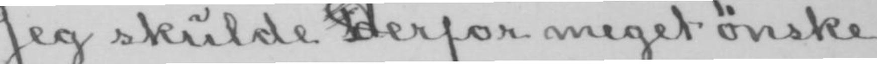Jeg skulde Dderfor meget ønske
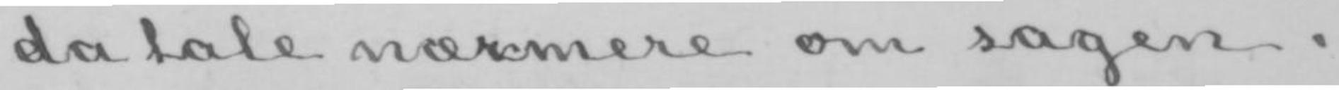da tale nærmere om sagen.
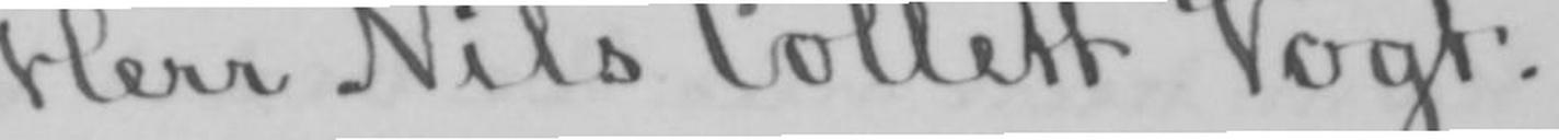Herr Nils Collett Vogt.
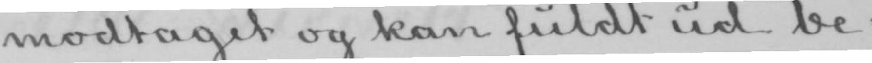modtaget og kan fuldt ud be-
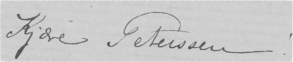Kjære Peterssen!
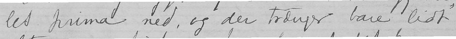let prima ned, og der trænger bare lidt
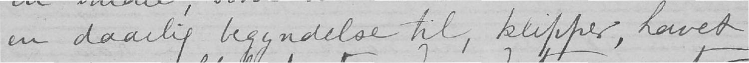en daarlig begyndelse til, klipper, havet
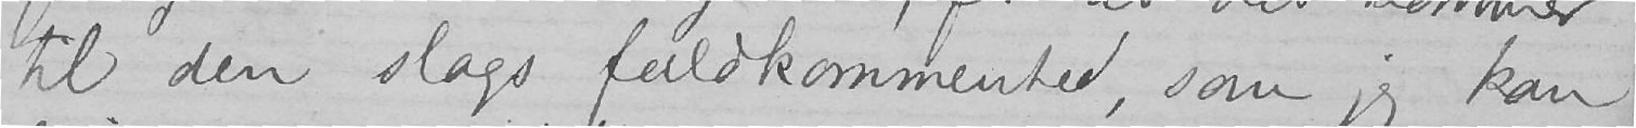til den slags fuldkommenhed, som jeg kan
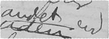andet end
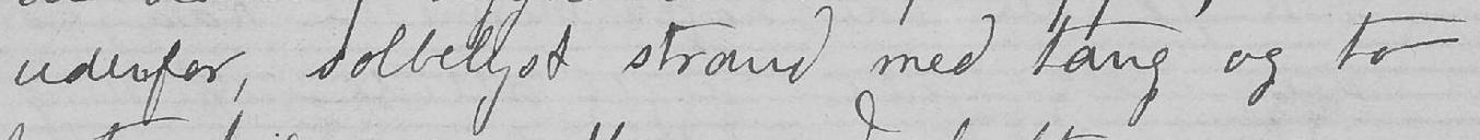udenfor, solbelyst strand med tang og to
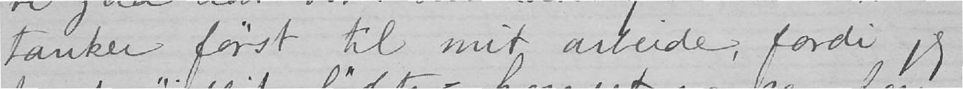tanker først til mit arbeide, fordi jeg
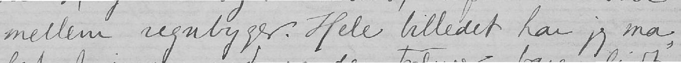mellem regnbyger. Hele billedet har jeg ma-
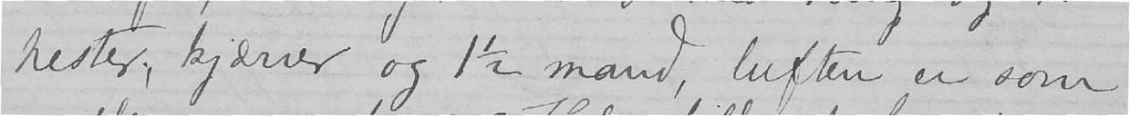hester, kjærrer og 1½ mand, luften er som
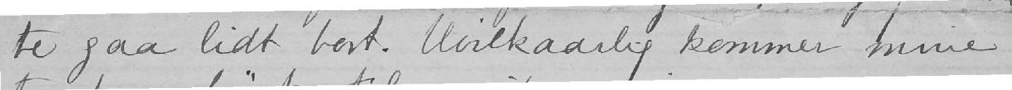te gaa lidt bort. Uvilkaarlig kommer mine
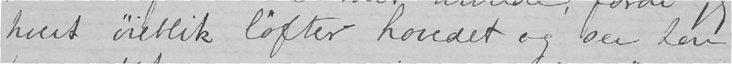hvert øieblik løfter hovedet og ser hen
